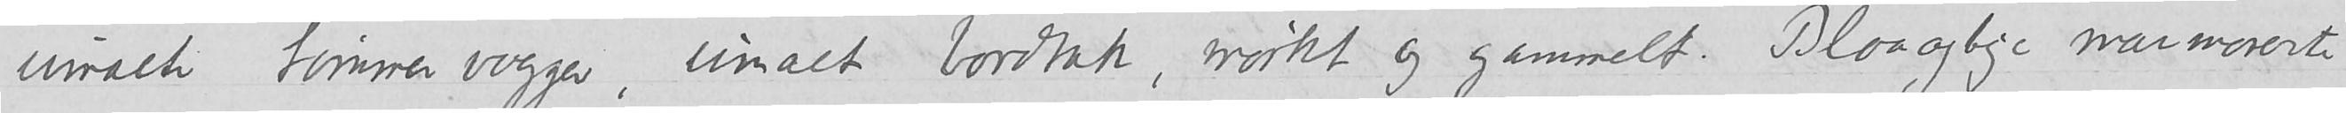umalte tømmervægger, umalt bordtak, mørkt og gammelt. Blaaagtige marmorerte
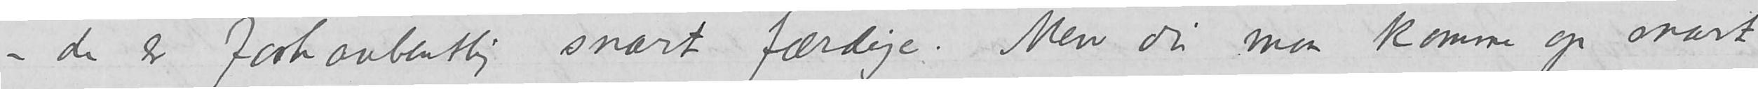– de er forhaabentlig snart færdige. Men du maa komme op snart
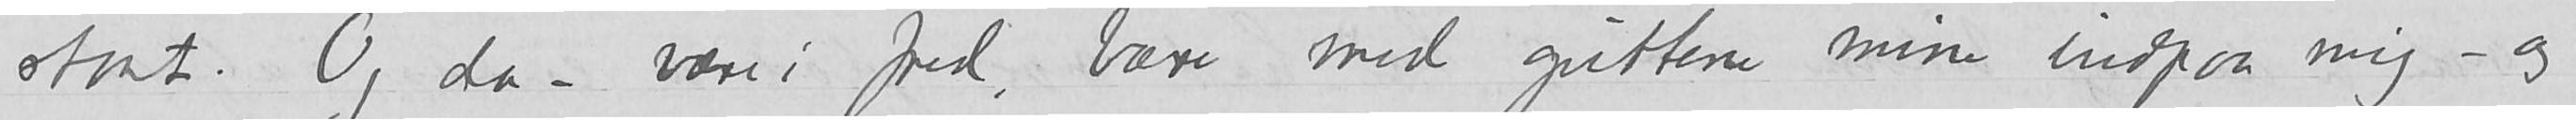staat. Og da – være i fred, bare med guttene mine indpaa mig – og
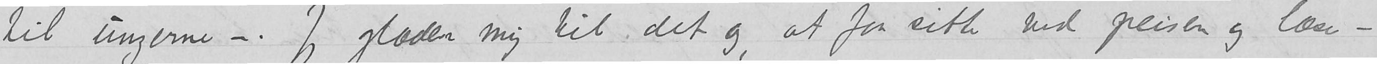til ungerne –. Jeg glæder mig til det og, at faa sitte ved peisen og læse –
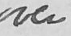over
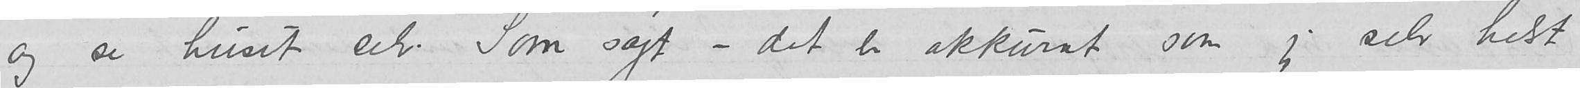og se huset selv. Som sagt – det er akkurat som jeg selv helst
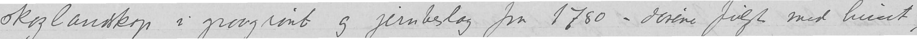skoglandskap i graagrønt og jernbeslag fra 1780 – dørene fulgte med huset,
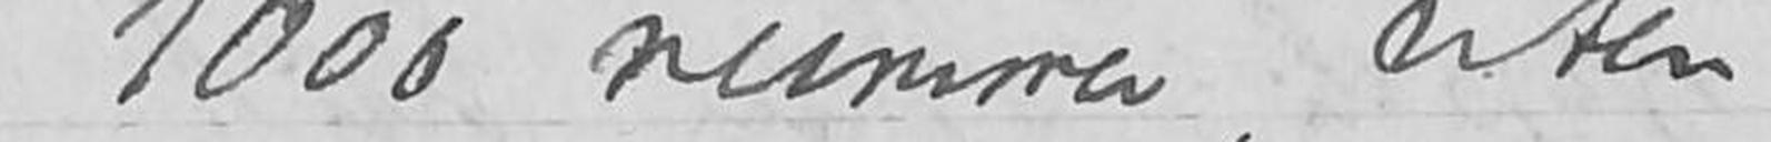1000 nummere, uten
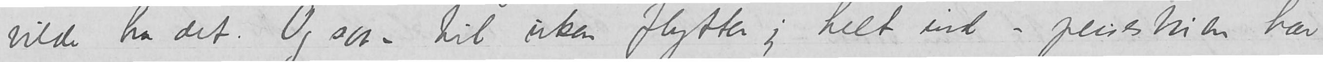vilda ha det. Og saa – til uken flytter jeg helt ind – peisestuen har
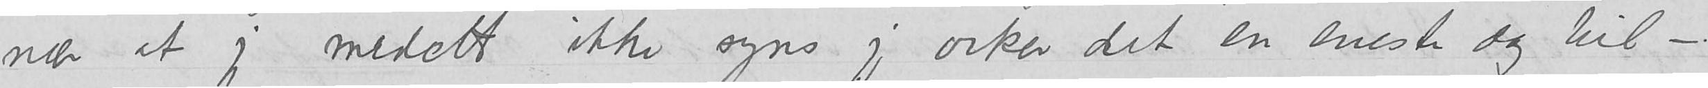nær at jeg medett ikke syns jeg orker det en eneste dag til –.
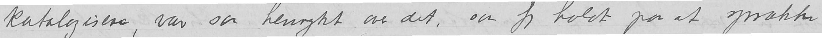katalogisere, var saa henrykt over det, saa jeg holdt paa at sprække
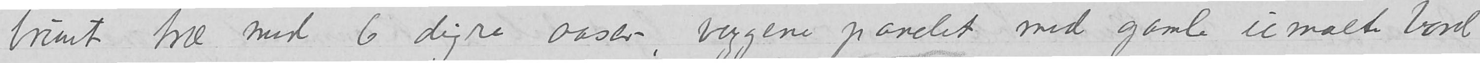brunt træ med 6 digre aaser, veggene panelet med gamle umalte bord
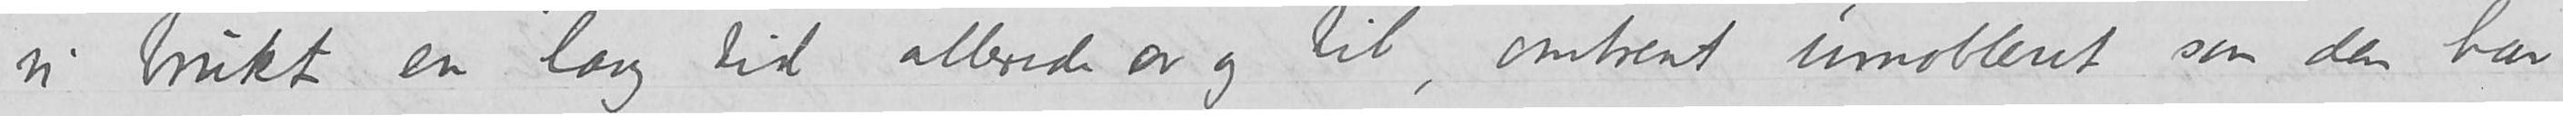vi brukt en lang tid allerede av og til, omtrent umøbleret som den har
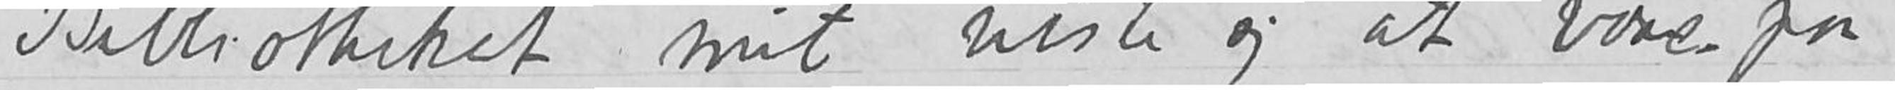Bibliotheket mit viste sig at være paa
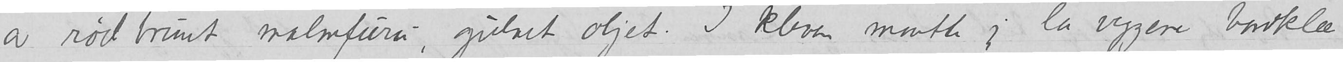av rødbrunt malmfuru, gulnet oljet. I kleven maatte jeg la veggene bordklæ
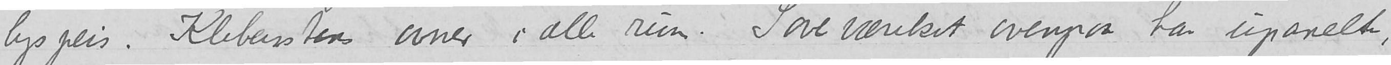lyspeis. Kleberstens ovner i alle rum. Soveværelset ovenpaa har upanelte,
